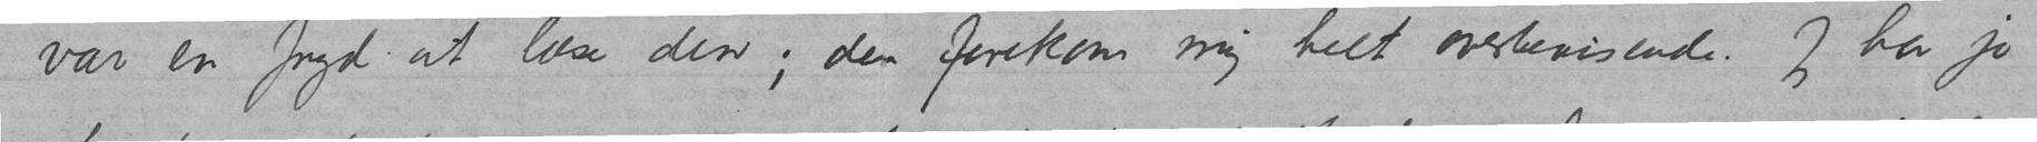var en fryd at læse den; den forekom mig helt overbevisende. Jeg har jo
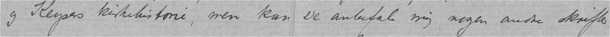og Keysers kirkehistorie, men kan De anbefale mig nogen andre skrifter
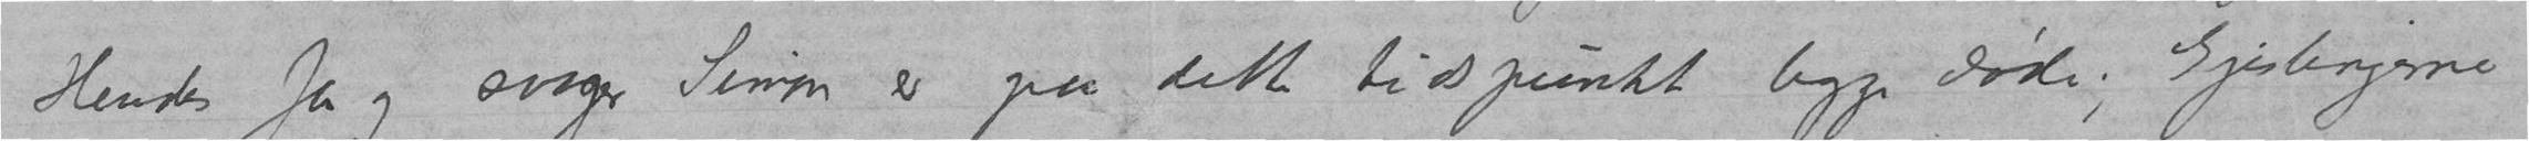Hendes far og svoger Simon er paa dette tidspunkt begge døde; Gjeslingerne
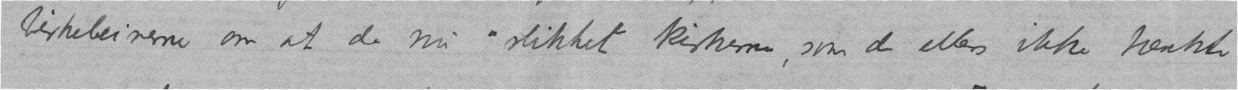birkebeinerne om at de nu "slikket kirkerne, som de ellers ikke tænkte
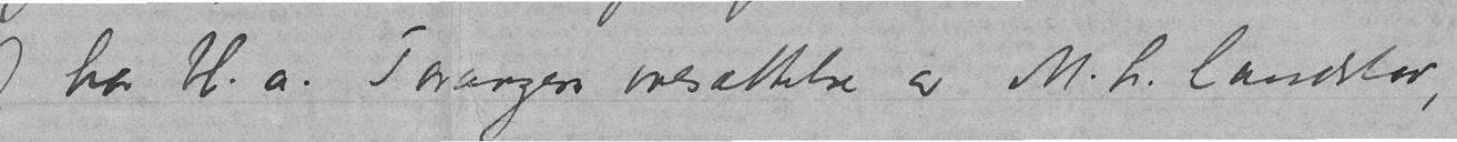– Jeg har bl.a. Tarangers oversættelse av M.L. landslov,
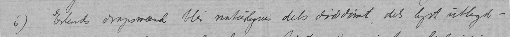6) Erlends drapsmænd blir naturligvis dels dødsdømt, dels lyst utlegd –
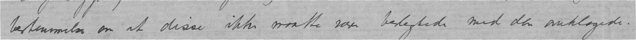bestemmelse om at disse ikke maatte være beslegtede med den anklagede.
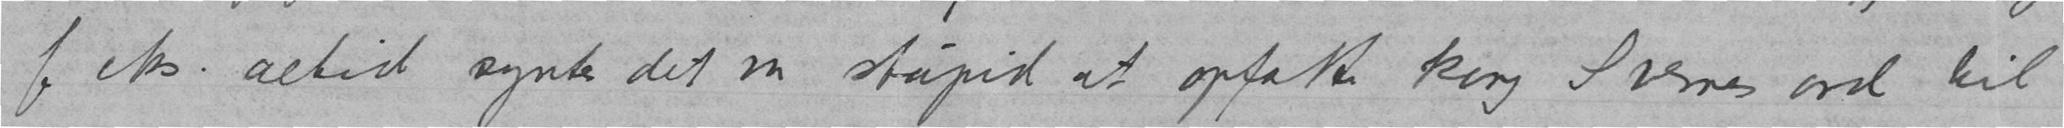f.eks. altid syntes det var stupid at opfatte kong Sverres ord til
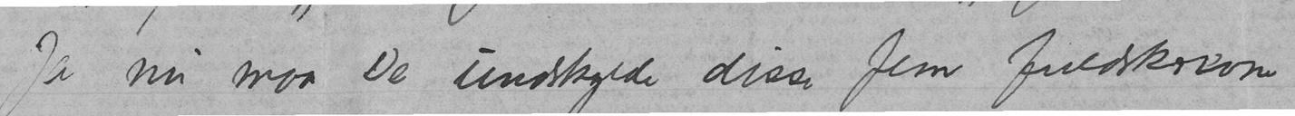Ja nu maa De undskylde disse fem fuldskrevne
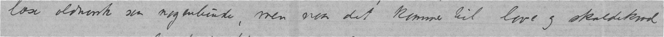læse oldnorsk saa nogenlunde, men naar det kommer til love og skaldekvad
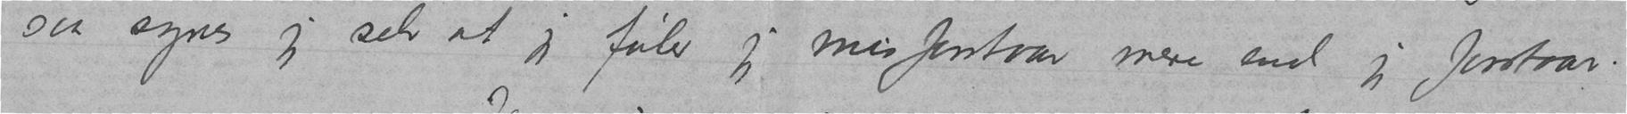saa synes jeg selv at jeg føler jeg misforstaar mere end jeg forstaar.
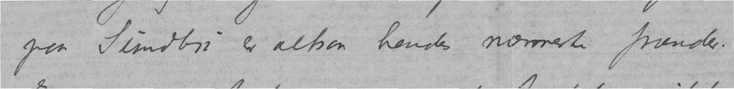paa Sundbø er altsaa hendes nærmeste frænder.
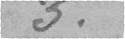3.
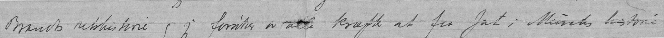Brandts retshistorie og jeg forsøker av alle kræfter at faa fat i Munchs historie
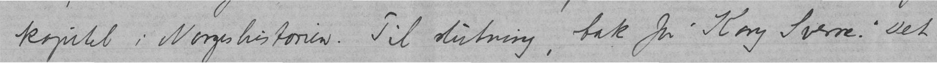kapitel i Norgeshistorien. Til slutning, tak for "Kong Sverre." Det
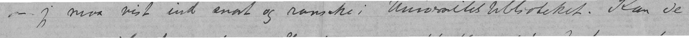– jeg maa vist ind snart og ransake i Universitesbiblioteket. Kan De
